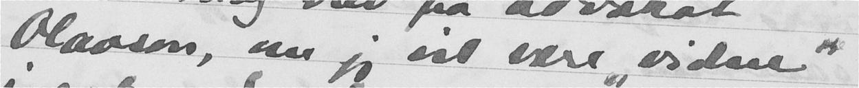Olavson, om jeg vil være "vidne"
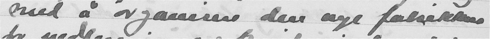med å organisere den nye fabrikken
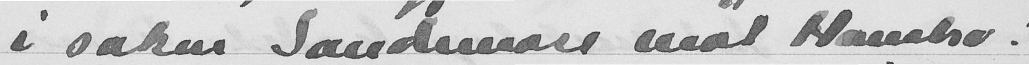i saken Sandemose mot Hambro!
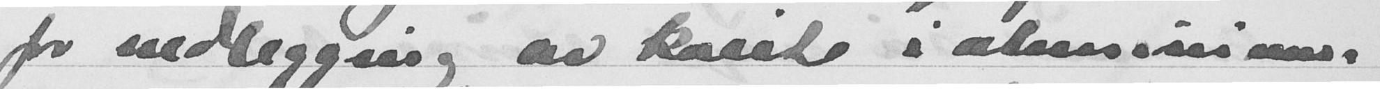for nedligging av kveite i aliminiums-
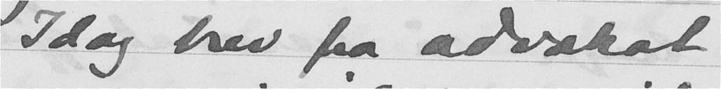I dag brev fra advokat
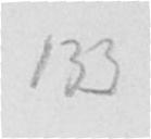133
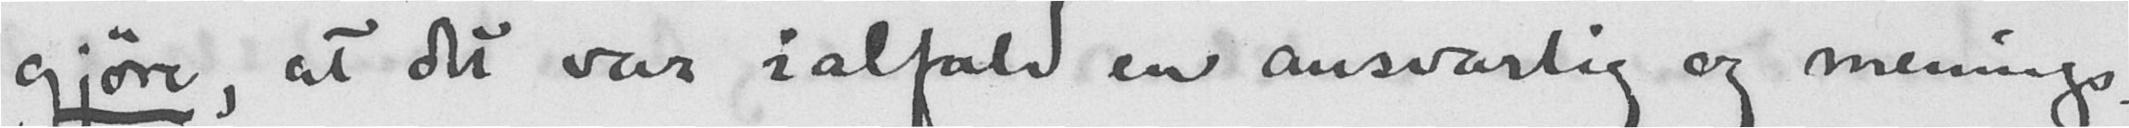gjøre, at det var ialfald en ansvarlig og menings-
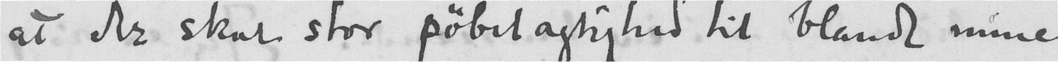at der skal stor pøbelagtighed til blandt mine
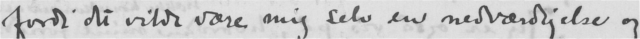fordi det vilde være mig selv en nedværdigelse og
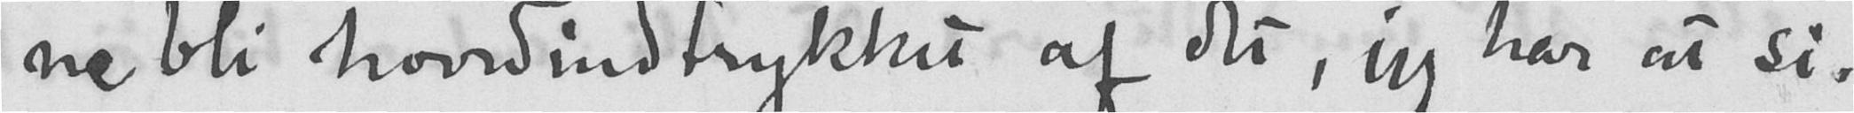ne bli hovedindtrykket af det, jeg har at si.
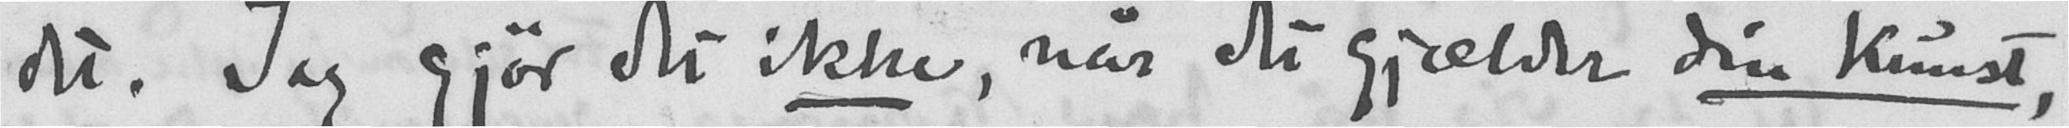det. Jeg gjør det ikke, når det gjælder din kunst,
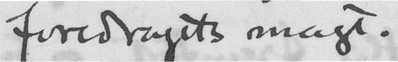foredragets magt.
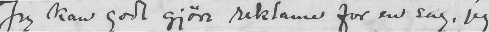Jeg kan godt gjøre reklame for en sag, jeg
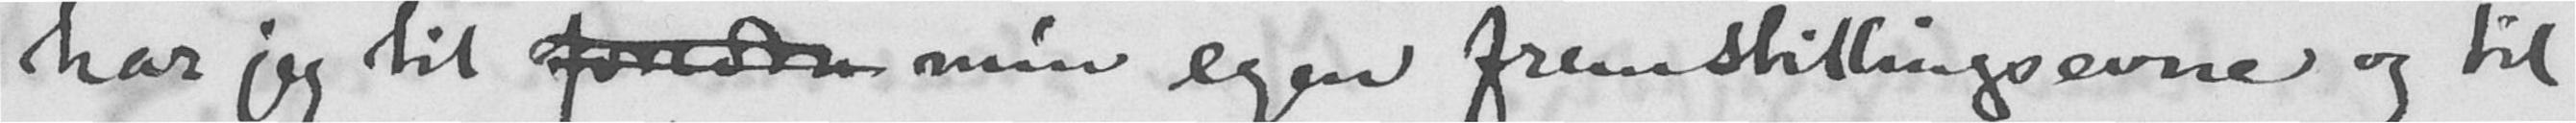har jeg til foredra min egen fremstillingsevne og til
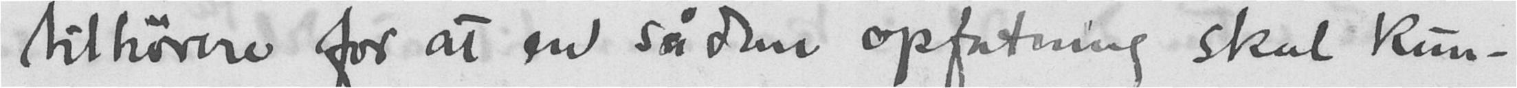tilhørere for at en sådan opfatning skal kun-
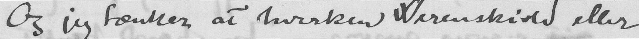Og jeg tænker at hverken Werenskiold eller
